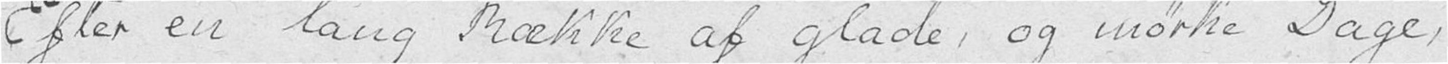Efter en lang Række af glade, og mørke Dage,
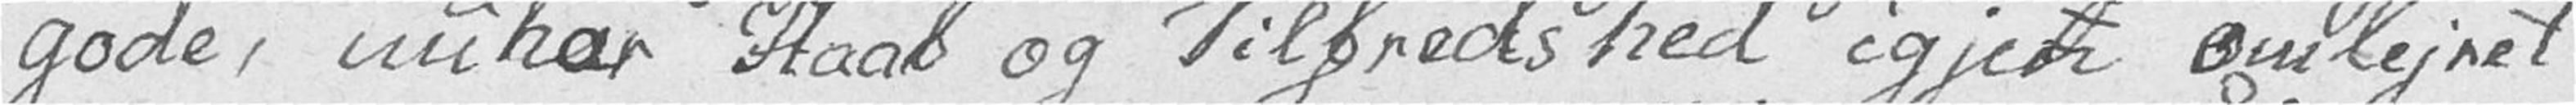gode, nu har Haab og Tilfredshed igjen omlejret
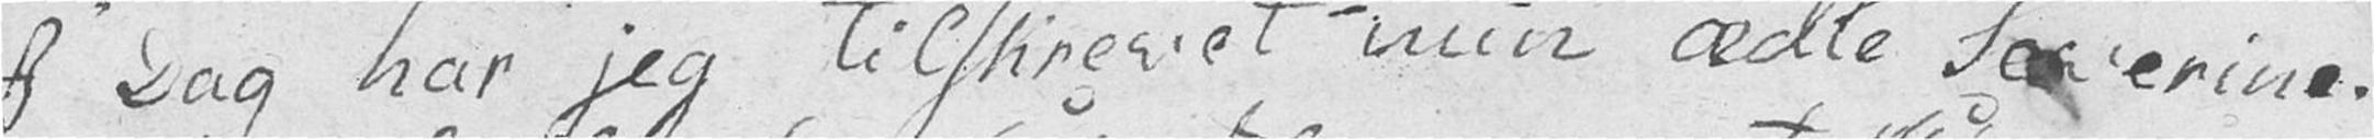I Dag har jeg tilskrevet min ædle Severine.
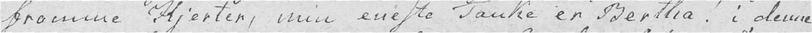fromme Hjerter, min eneste Tanke er Bertha! i denne
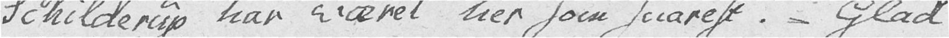Schilderup har været her som snarest. – Glad
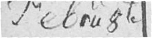Febru 8te
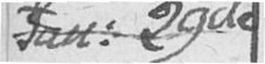Jan. 29de
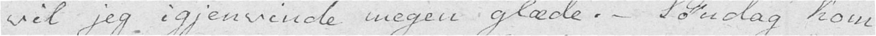vil jeg igjenvinde megen glæde. – Søndag kom
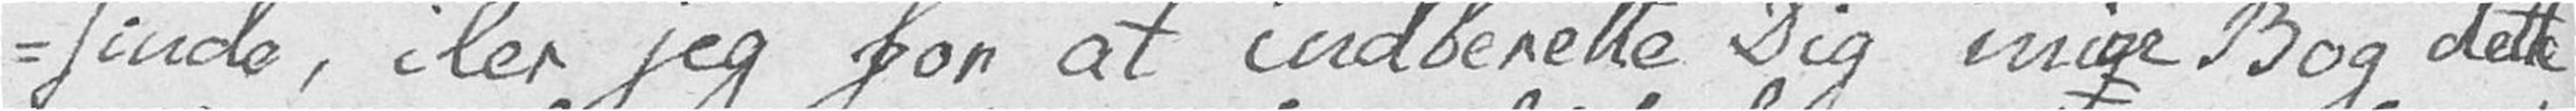-sinde, iler jeg for at indberette Dig min Bog dette
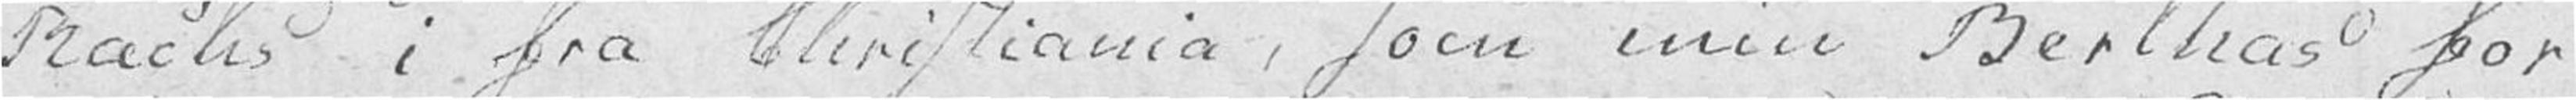Rachs i fra Christiania, som min Berthas for
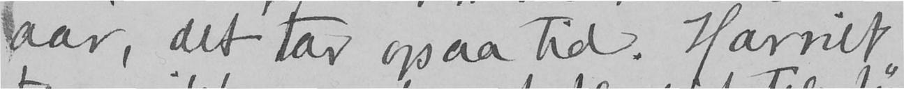aar, det tar ogsaa tid. Harriet
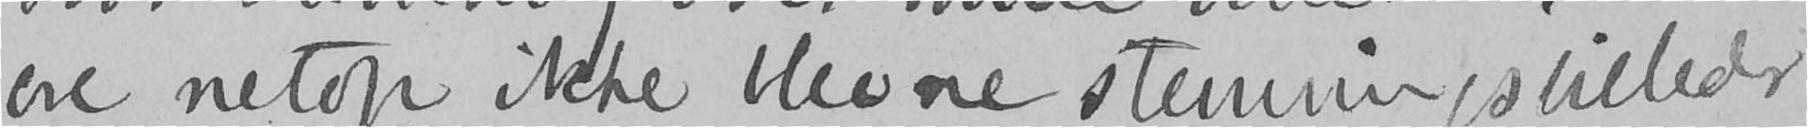ere netop ikke blevne stemningsbilleder
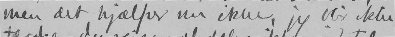men det hjælper nu ikke, jeg blir ikke
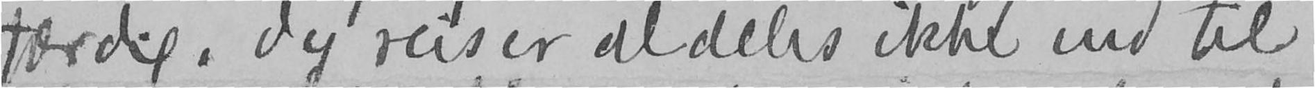færdig. Jeg reiser aldeles ikke ind til
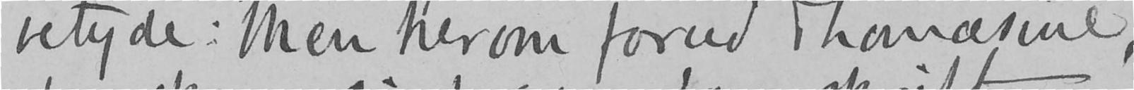betyde. Men herom forud Thomasine,
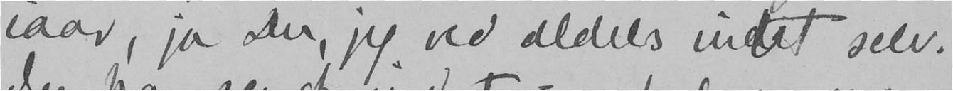iaar, ja Du, jeg ved aldeles intet selv.
